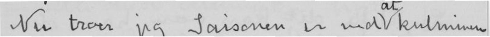Nu tror jeg Saisonen er med at kulminere,
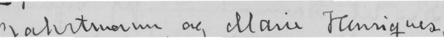Jahrtmann, og Marie Henniques
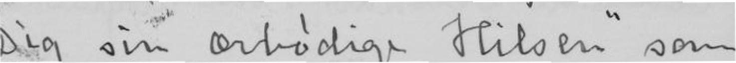Dig sin "ærbødige Hilsen", som
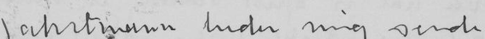Jahrtmann beder mig sende
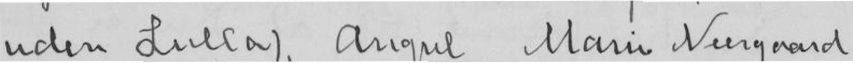uden Lulla), Angul, Marie Neergaard
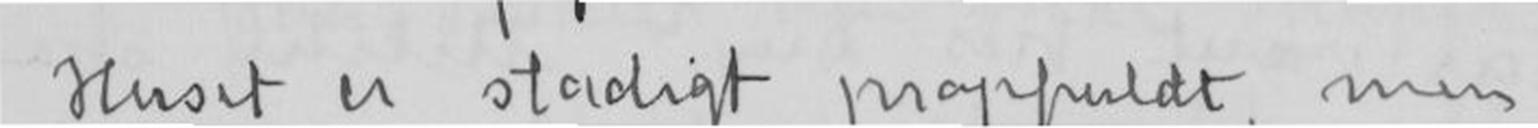Huset er stadig propfuldt, men
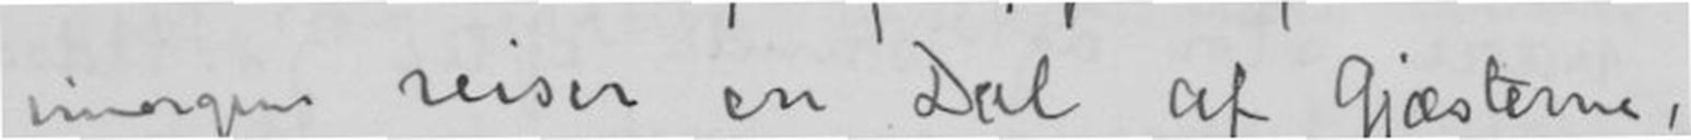imorgen reiser en Del af gjæstene,
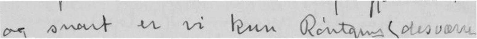og snart er vi kun Röntgens, (desværre
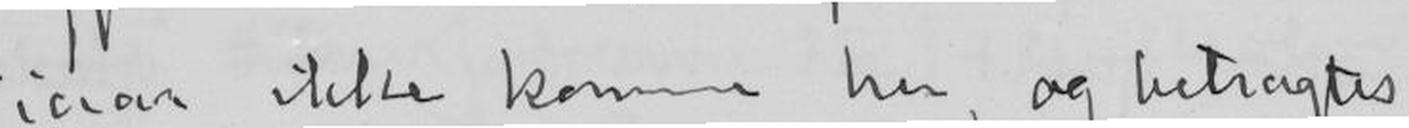iaar ikke komme her, og betragtes
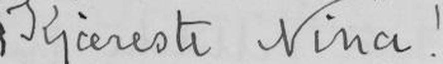Kjæreste Nina!
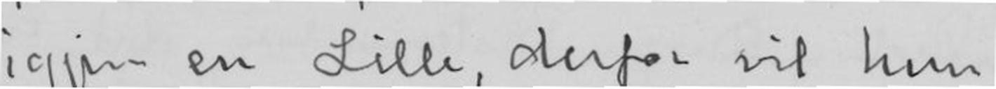igjen en Lille, derfor vil hun
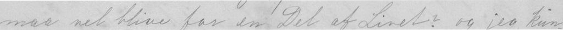maa vel blive for en Del af Livet? og jeg kun-
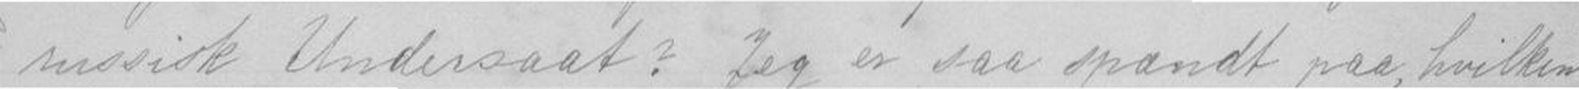russisk Undersaat? Jeg er saa spændt paa, hvilken
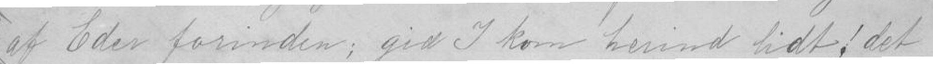af Eder forinden; gid I kom hevind Lidt; det
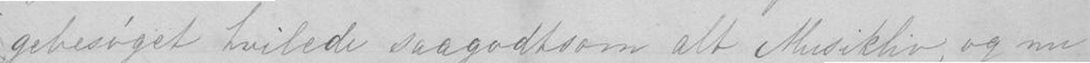gebesøget hvilede saagodtsom alt Musikliv, og mu
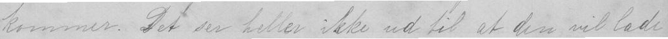kommer. Det ser heller ikke ud til at den vil lade
